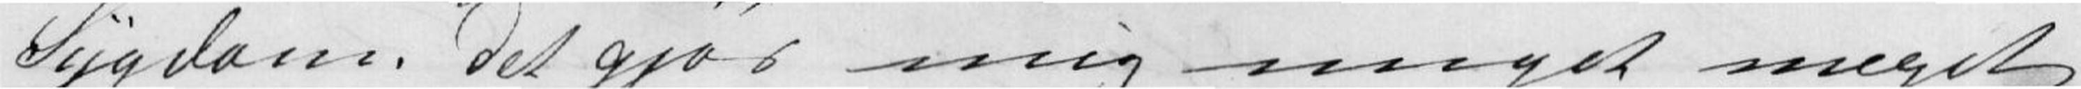Sygdom. Det gjør mig meget meget
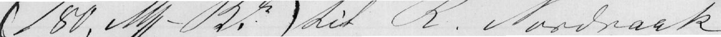(180,M-B.) til R. Nordraak
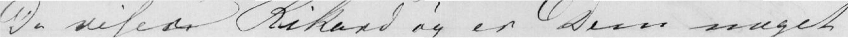Da viser Rikard og er Dem meget
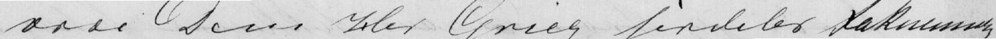være Dem Herr Grieg serdeles taknemmelig
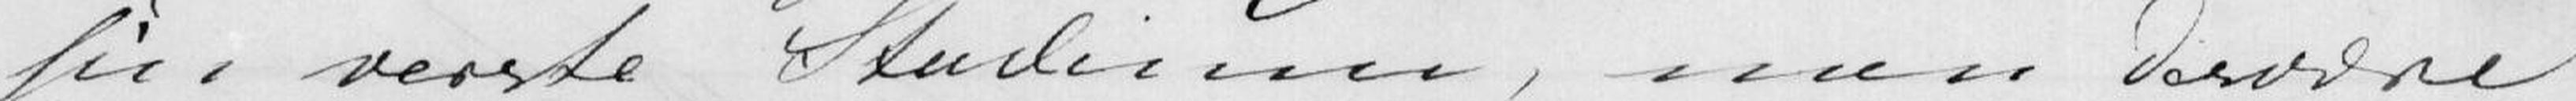sin verste Stadium, men desværre
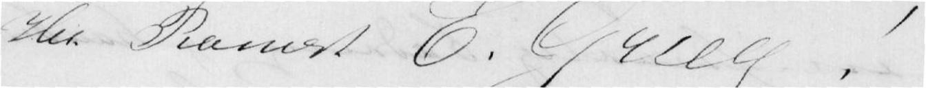Herr Pianist E.Grieg!
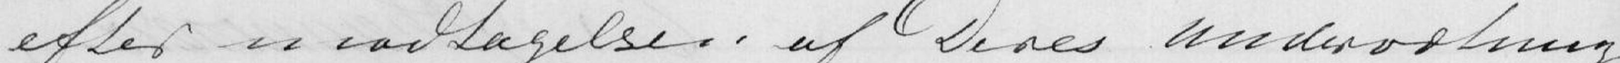efter modtagelsen af Deres underrætning
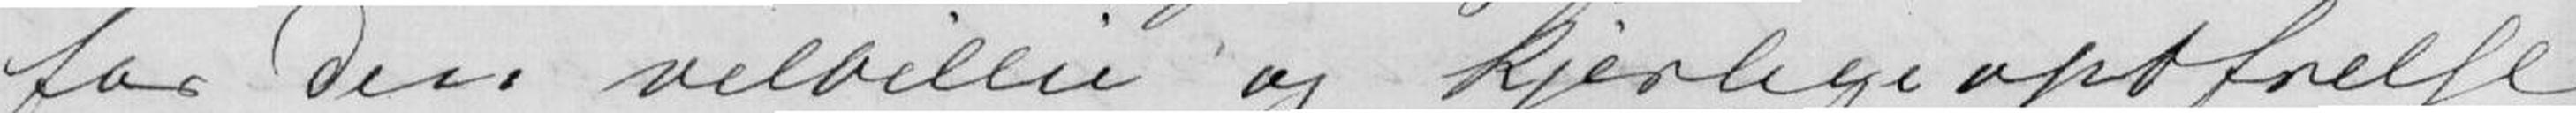for Den velvillie og kjerlege opofrelse
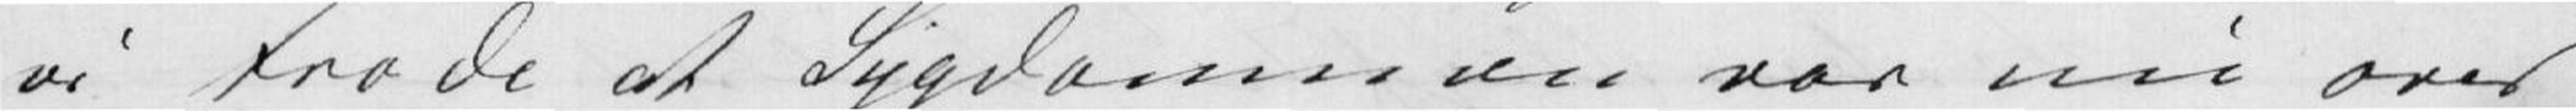vi trode at Sygdommen var nu over
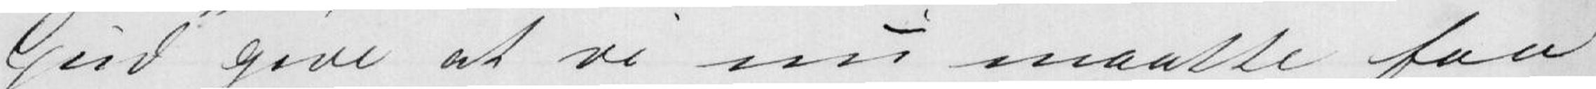Gud give at vi nu maatte faa
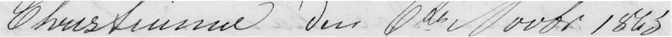Christiania den 6de Novb 1875
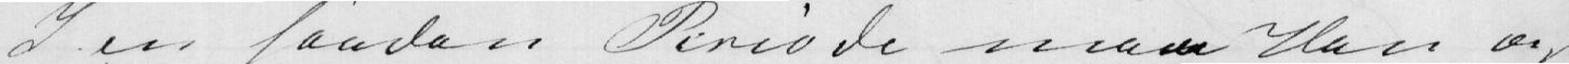I en saadan Periode maa Han og
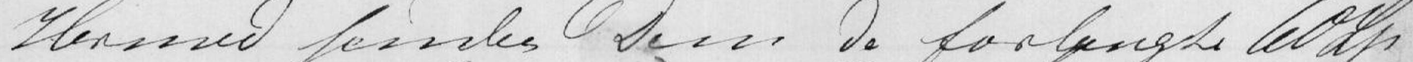Hermed sendes Dem De forlangte 60Sp
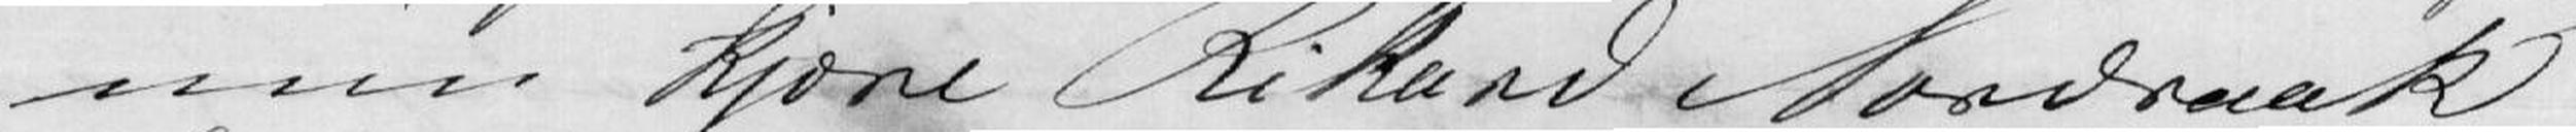min kjære Rikard Nordraak
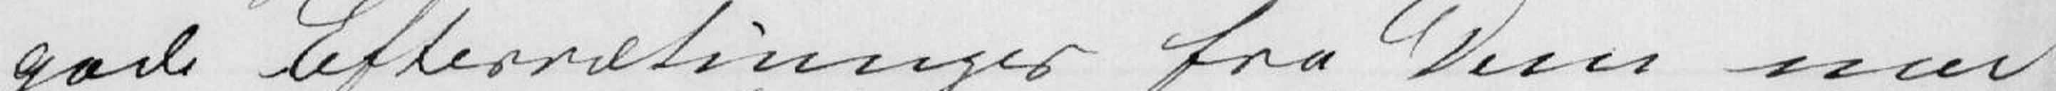god Efterrætninger fra Dem med
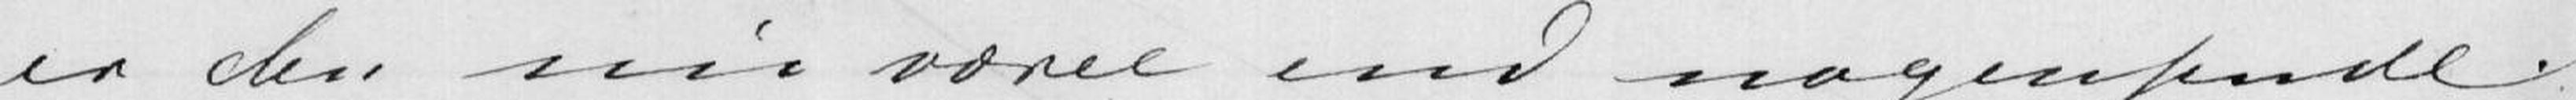er der nu værre end nogensinde.
